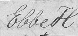Ebbe H.
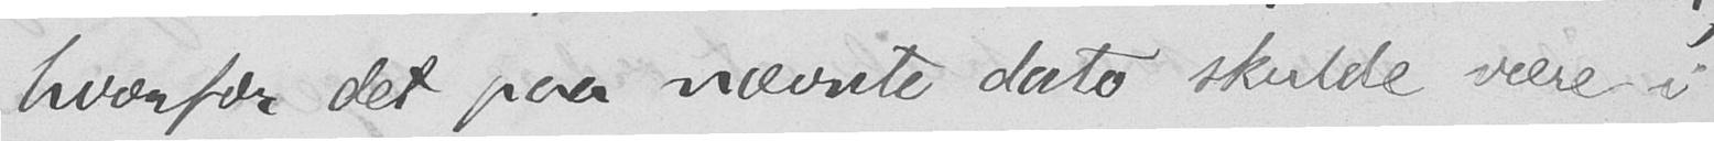hvorfor det paa nævnte dato skulde være i
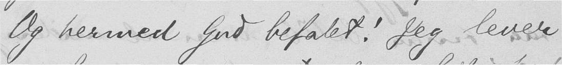Og hermed Gud befalet! Jeg lever
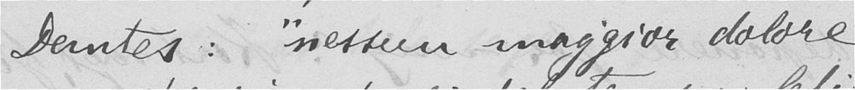Dantes: "nessun maggior dolore
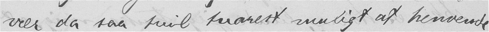vær da saa snil snarest muligt at henvende
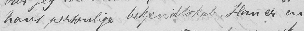hans personlige bekjendtskab. Han er en
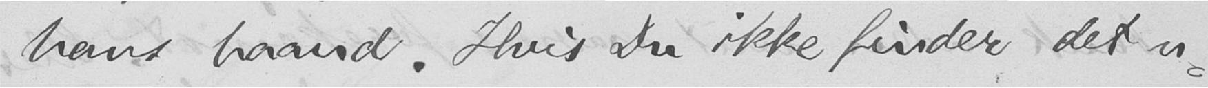hans haand. Hvis Du ikke finder det u-
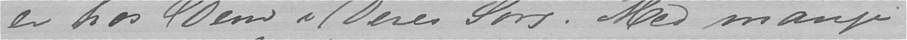er hos Dem i Deres Sorg. Med mange
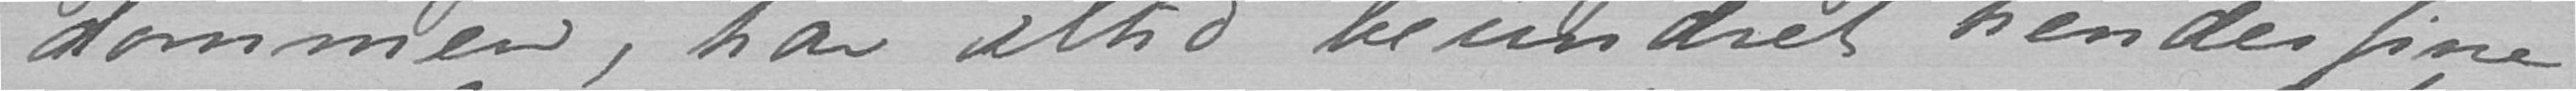dommen, har altid beundret hendes fine
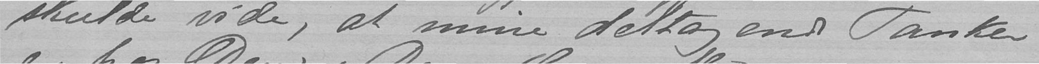skulde vide, at mine deltagende Tanker
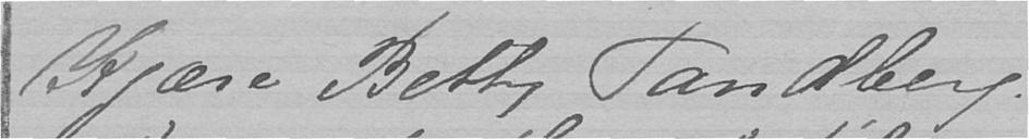Kjære Betty Tandberg.
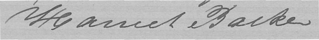Harriet Backer
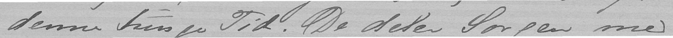denne tunge Tid. De deler Sorgen med
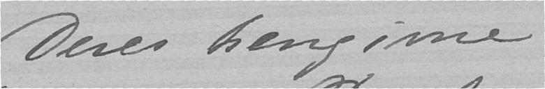Deres hengivne
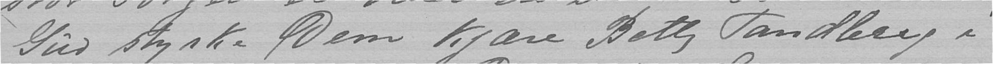Gud styrke Dem Kjære Betty Tandberg i
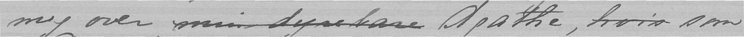mig over min dyrebare Agathe, hvis som
